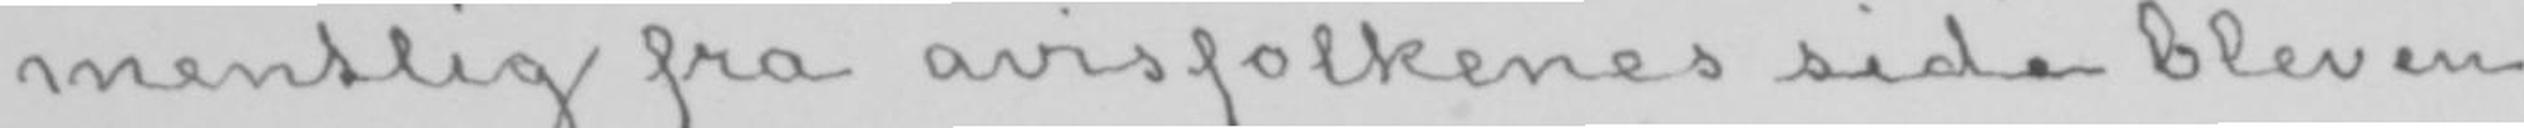mentlig fra avisfolkenes side bleven
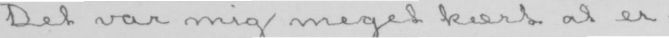Det var mig meget kært at er-
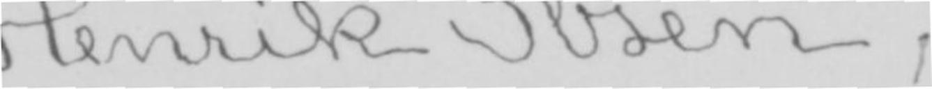Henrik Ibsen.
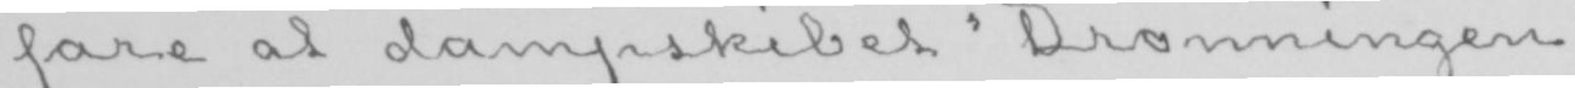fare at dampskibet «Dronningen»
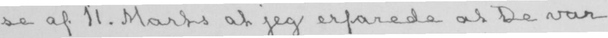se af 11. Marts at jeg erfarede at De var
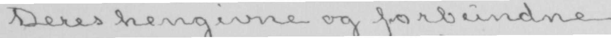Deres hengivne og forbundne
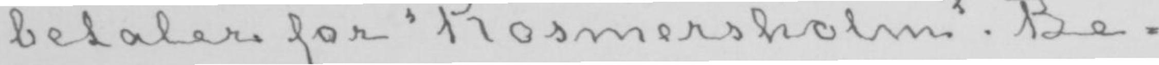betaler for «Rosmersholm». Be-
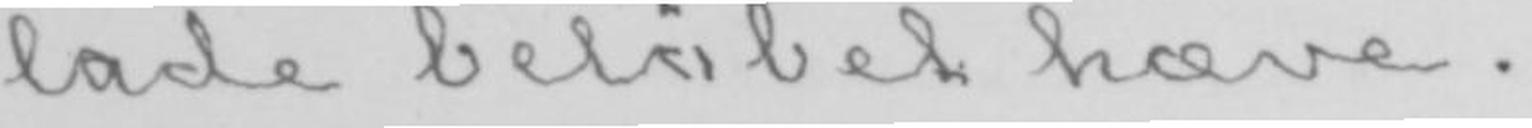lade beløbet hæve.
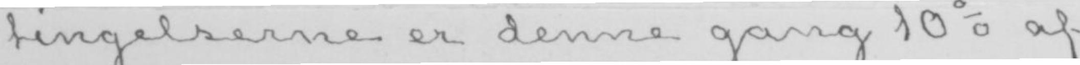tingelserne er denne gang 10 &percnt; af
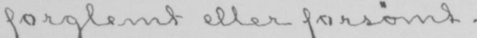forglemt eller forsømt.
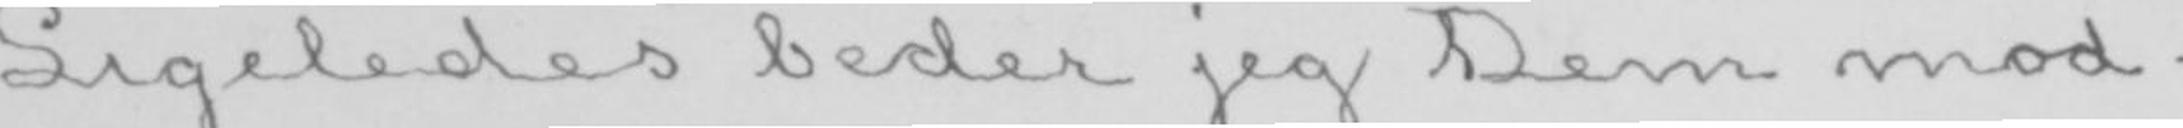Ligeledes beder jeg Dem mod-
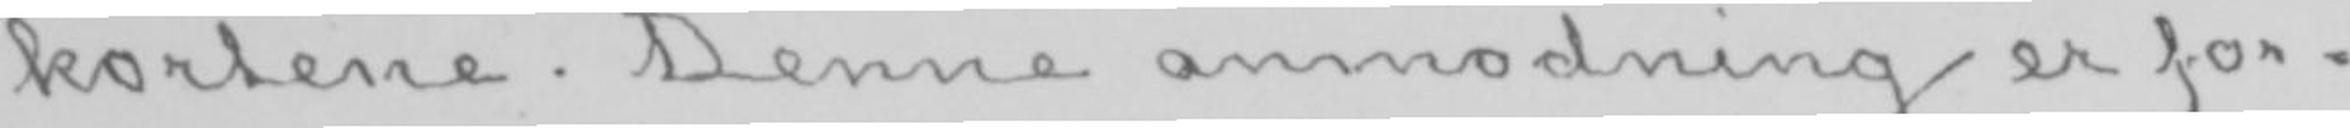kortene. Denne anmodning er for-
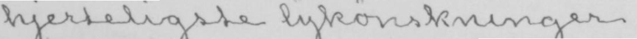hjerteligste lykønskninger.
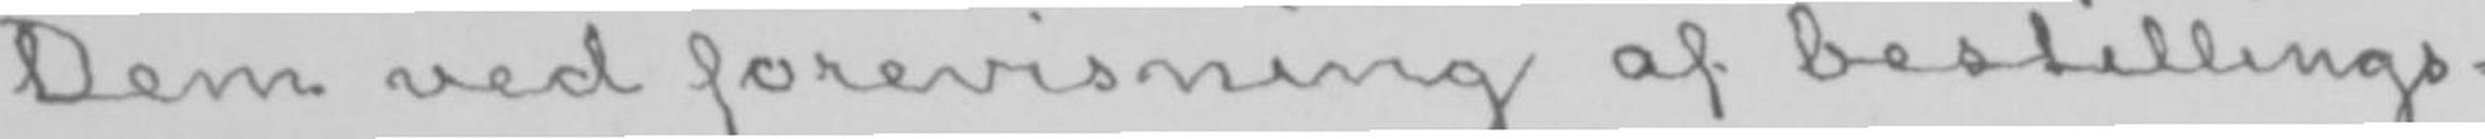Dem ved forevisning af bestillings-
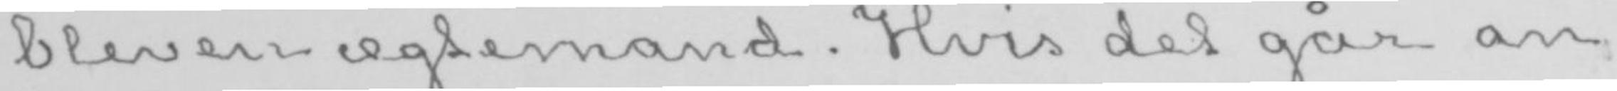bleven ægtemand. Hvis det går an
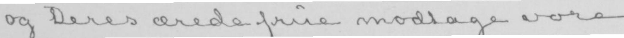og Deres ærede frue modtage vore
